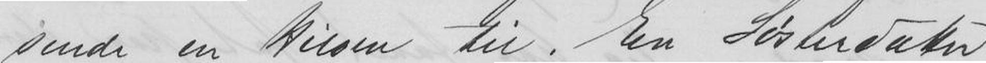sende en Hilsen til. En Søsterdatter
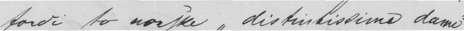fordi to norske distintissime dame
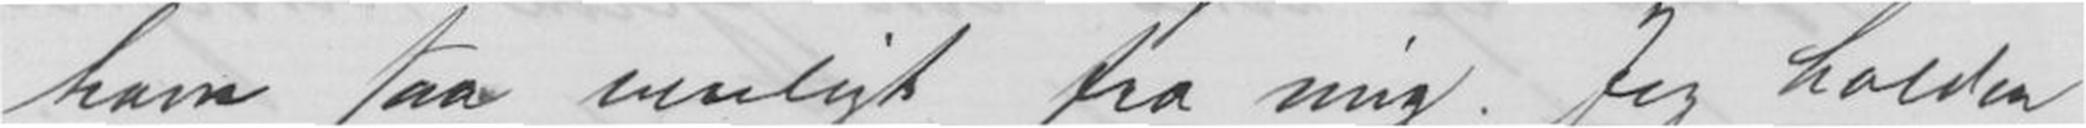ham saa venligt fra mig. Jeg holder
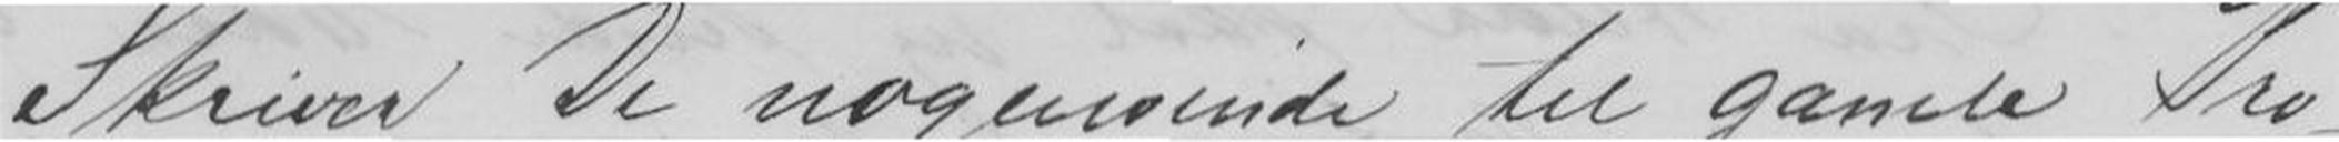Skriver De nogensinde til gamle Pro-
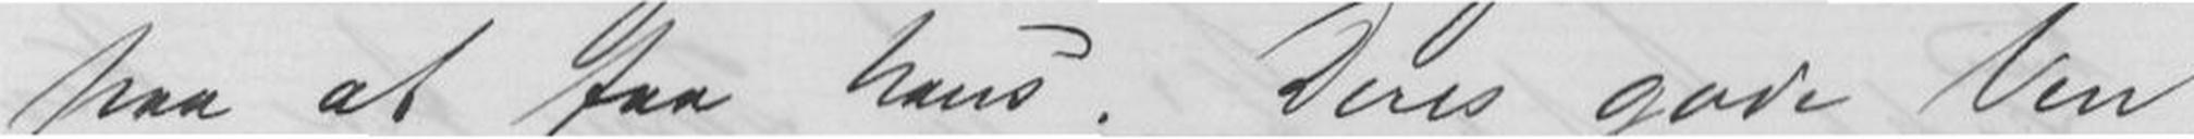paa at faa hans. Deres gode Ven
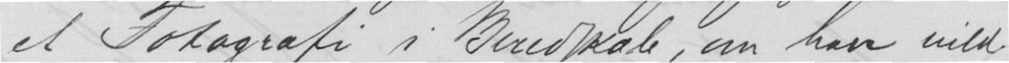et Fotografi i Beredskab, om han vilde
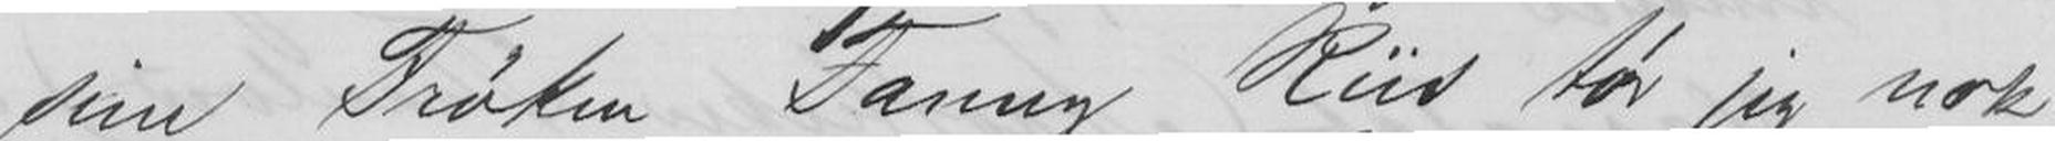sine Frøken Fanny Riis tør jeg nok
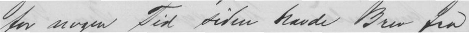for nogen Tid siden havde Brev fra,
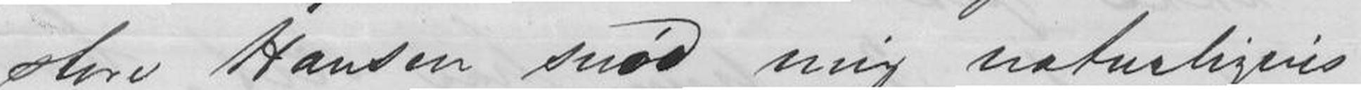store Hansen snød mig naturligvis
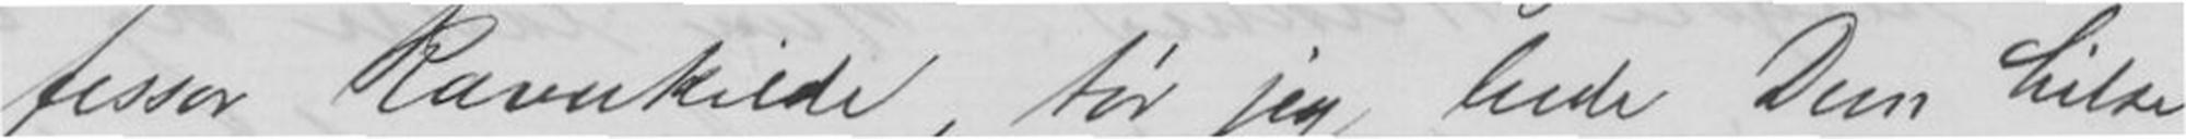fessor Ravnkilde, tør jeg bede Dem hilse
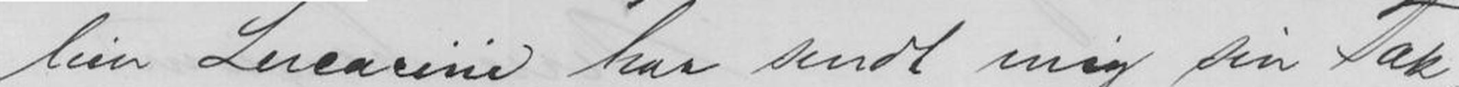lien Lercarini har sendt mig sin Tak,
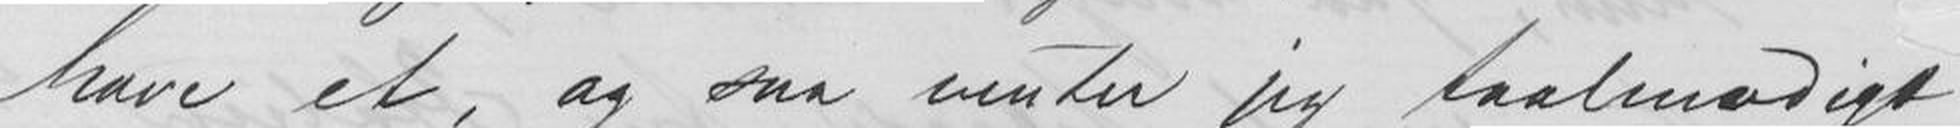have et, og saa venter jeg taalmodigt
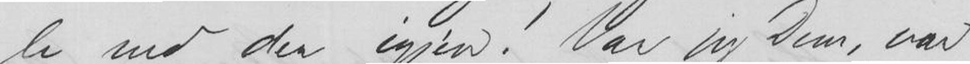le ned der igjen! Var jeg Dem, var
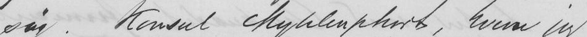søg. Konsul Myklenphort, hvem jeg
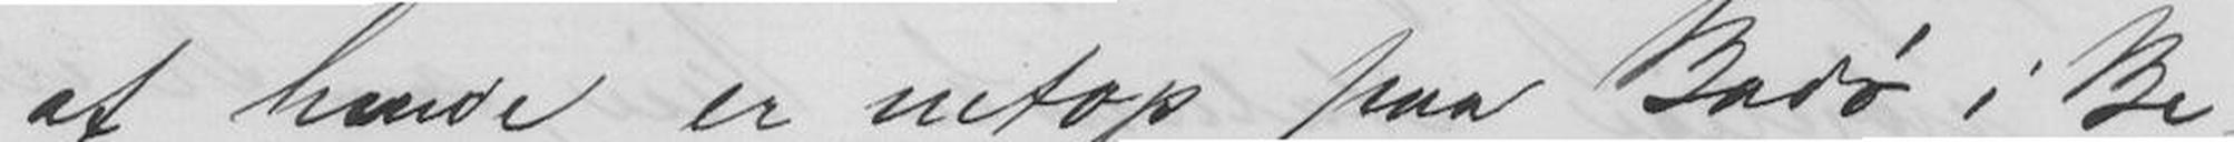af hende er netop paa Bodø i Be-
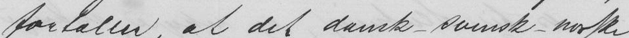fortæller, at det dansk-svensk-norske
